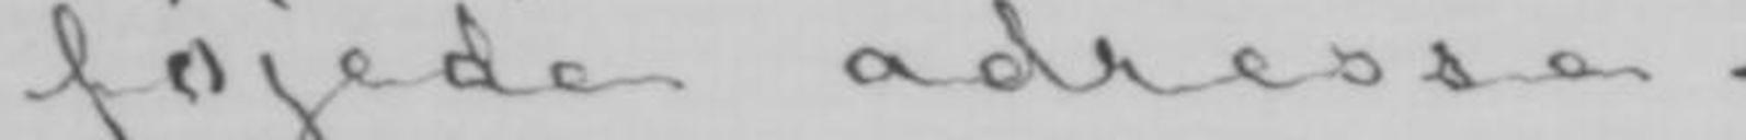føjede adresse.
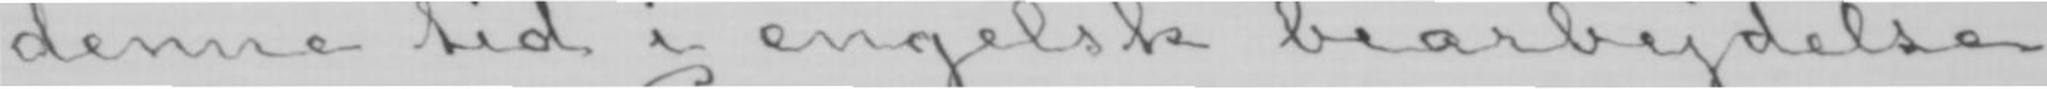denne tid i engelsk bearbejdelse
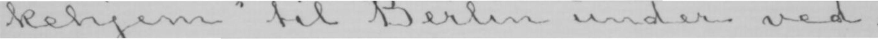kehjem» til Berlin under ved-
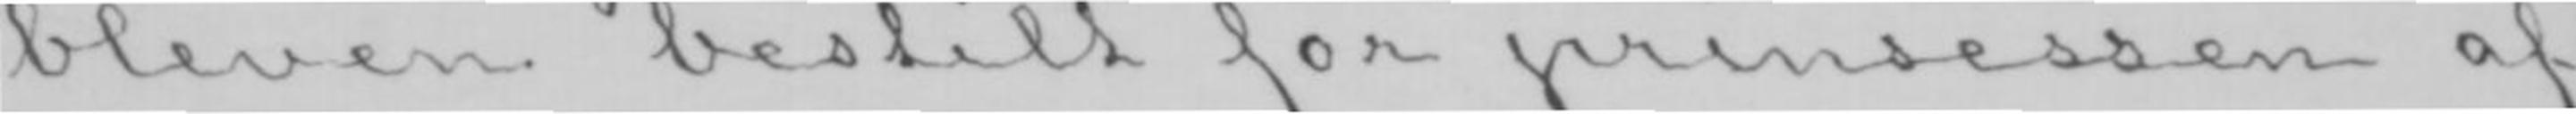bleven bestilt for prinsessen af
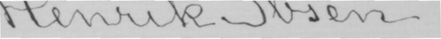Henrik Ibsen.
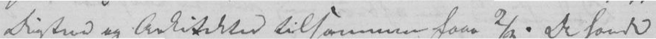Digtere og Arkitekter tilsammen faar 2/3. De havde
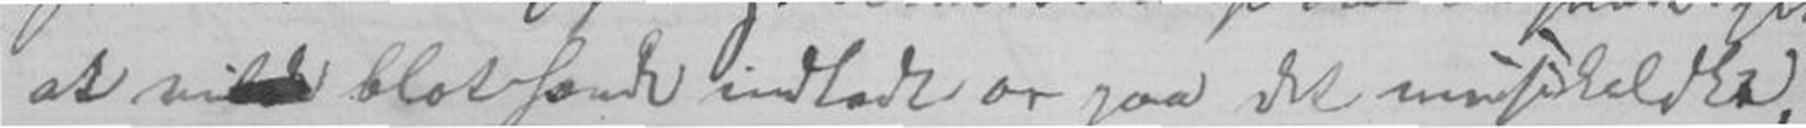at vi blot havde indlade os paa det musikalske,
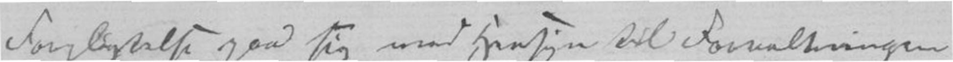Forpligtelse gav sig med hensyn til Forvaltningen
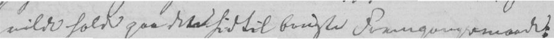vilde holde paa den hidtil brugte Fremgangsmaade:
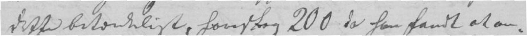dette betænkeligt, foreslog 200 da han fandt at
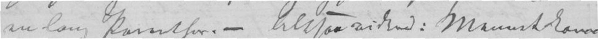lang Paranthes. - Altsaa videre: Manust kom
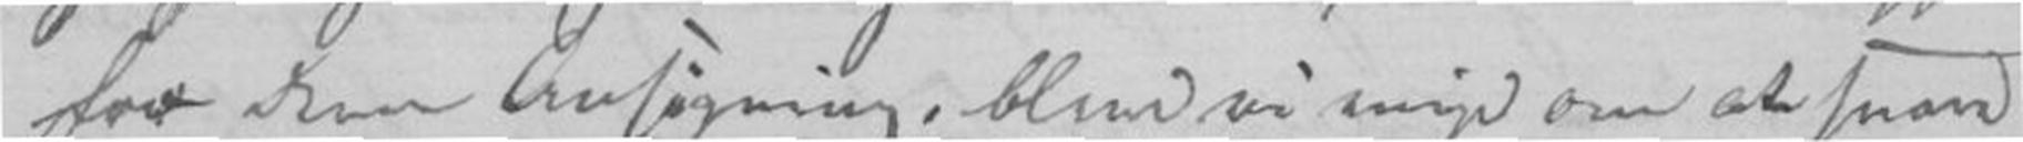for Deres Ansøgning, blev vi enige om at snart
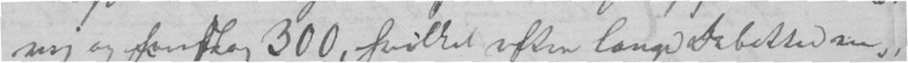vej og foreslog 300, hvilket efter lange Debatter en-
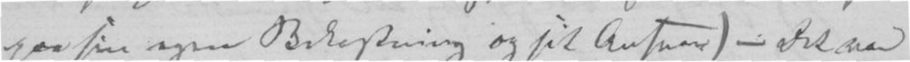paa sin egen Bekostning og sit Ansvar) - Det var
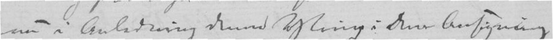nu i Anledning denne Yting i Deres Ansøkning
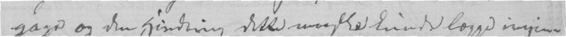gage og den Hindring dette maaske kunde lægge ivejen
